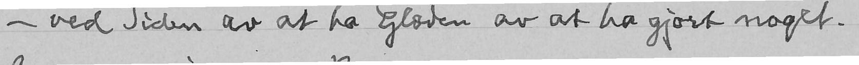- ved Siden av at ha Glæden av at ha gjort noget.
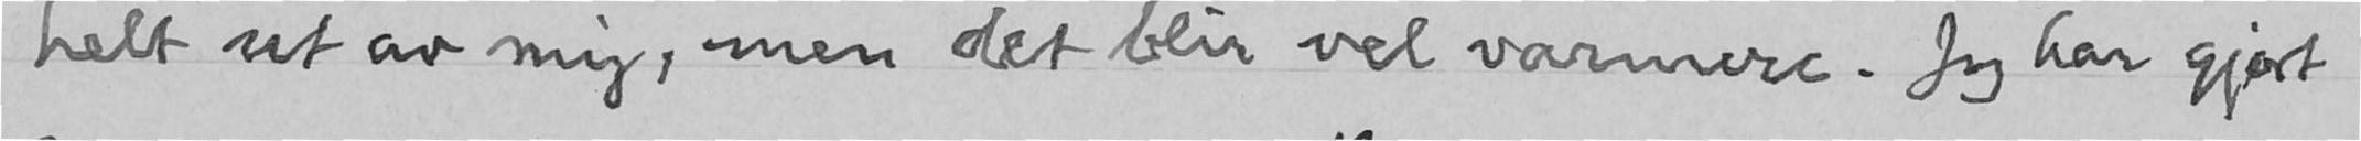helt ut av mig, men det blir vel varmere. Jeg har gjort
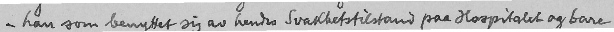- han som benyttet sig av hendes Svakhetstilstand paa Hospitalet og bare
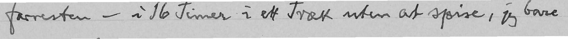forresten - i 16 Timer i ett Træk uten at spise, jeg bare
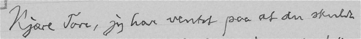Kjære Tore, jeg har ventet paa at du skulde
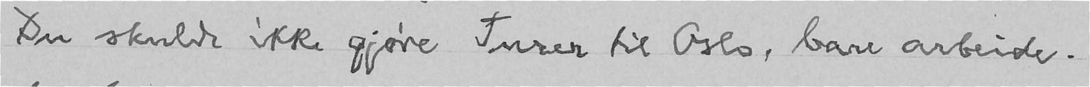Du skulde ikke gjøre Turer til Oslo, bare arbeide.
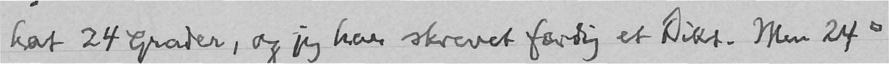hat 24 Grader, og jeg har skrevet færdig et Dikt. Men 24 º
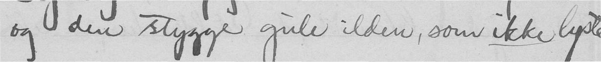og den stygge gule ilden, som ikke lyste
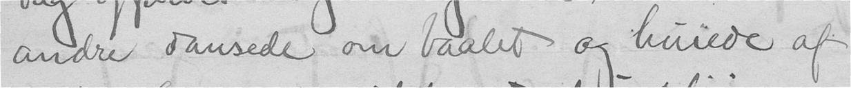andre dansede om baalet og huiede af
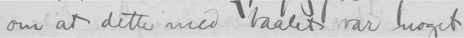om at dette med baalet var noget
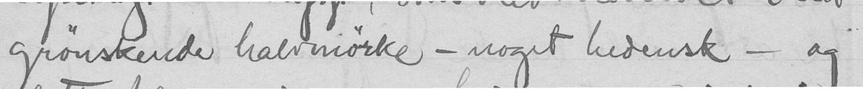grønskende halvmørke, - noget hedensk - og
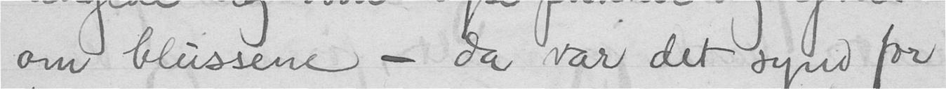om blussene - da var det synd for
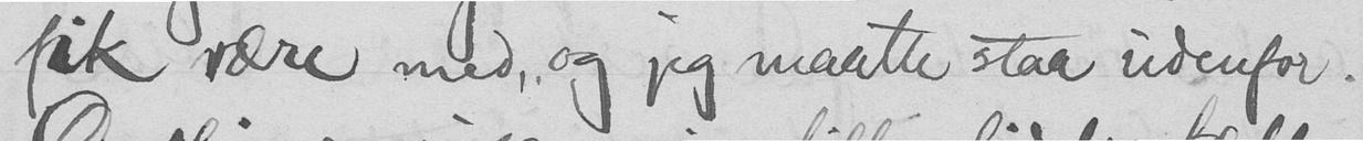fik være med, og jeg maatte staa udenfor.
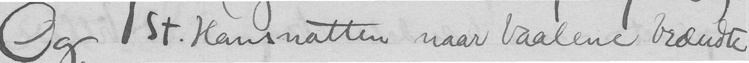Og st. Hansnatten naar baalene brændte
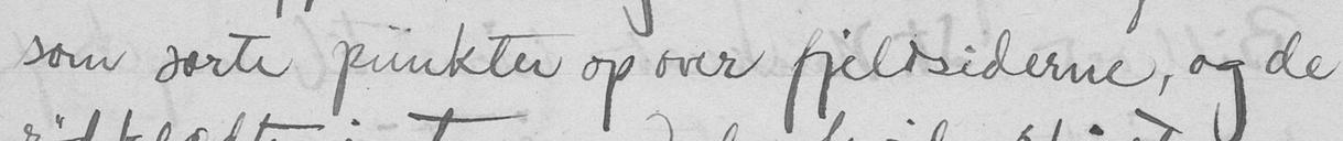som sorte punkter op over fjeldsiderne, og de
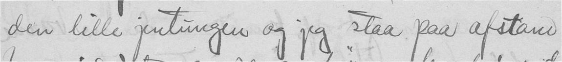den lille jentungen og jeg staa paa afstand
